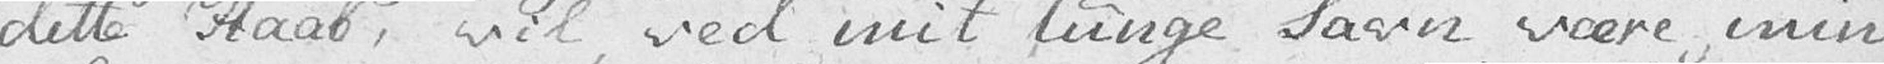dette Haab, vil ved mit tunge Savn være min
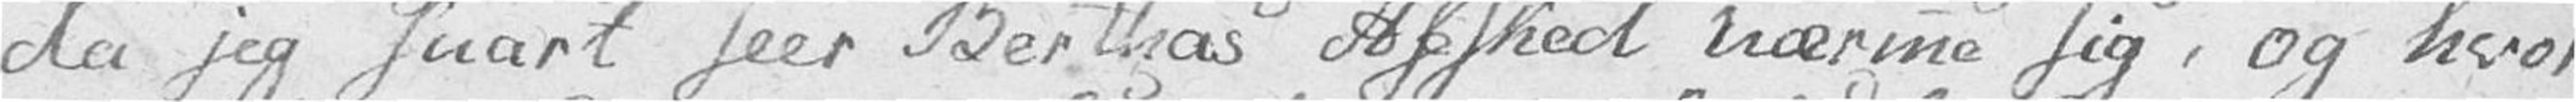da jeg snart seer Berthas Afsked nærme sig, og hvor
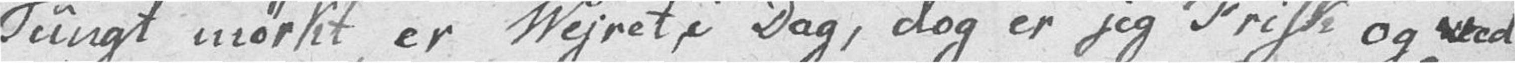Tungt mørkt er Vejret i Dag, dog er jeg Frisk og ved
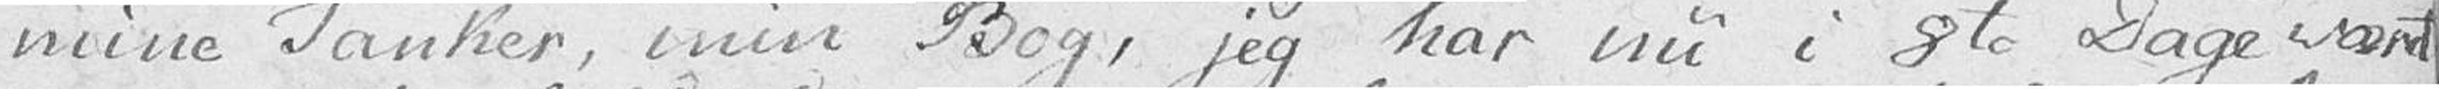mine Tanker, min Bog, jeg har nu i 8te Dage vært
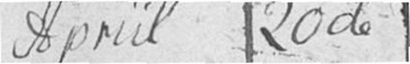Apriil 20de
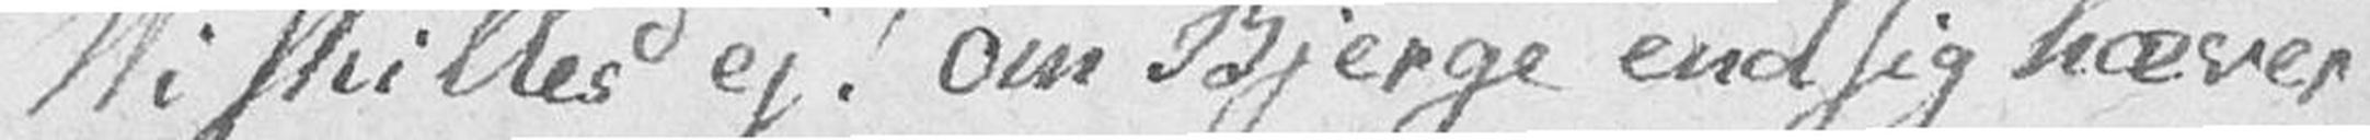Vi skilles ej! om Bjerge end sig hæver
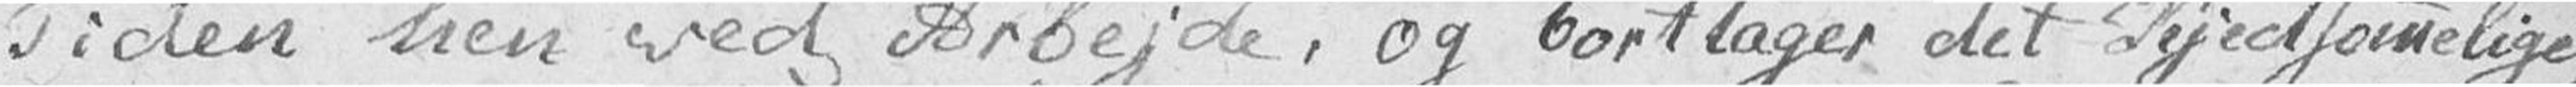Tiden hen ved Arbejde, og borttager det Kjedsommelige,
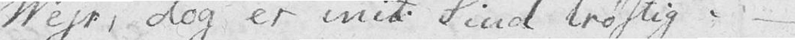Vejr, dog er mit Sind Trøstig. –
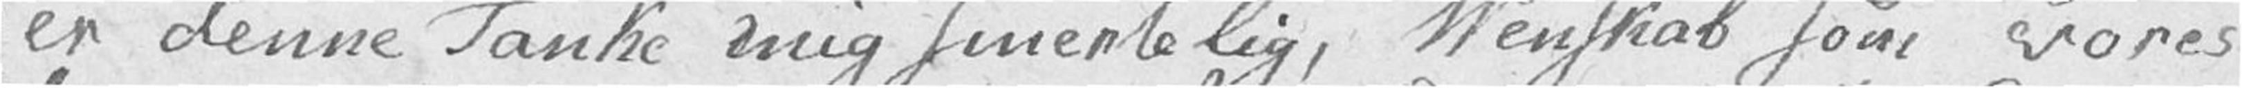er denne Tanke mig smertelig, Venskab som vores
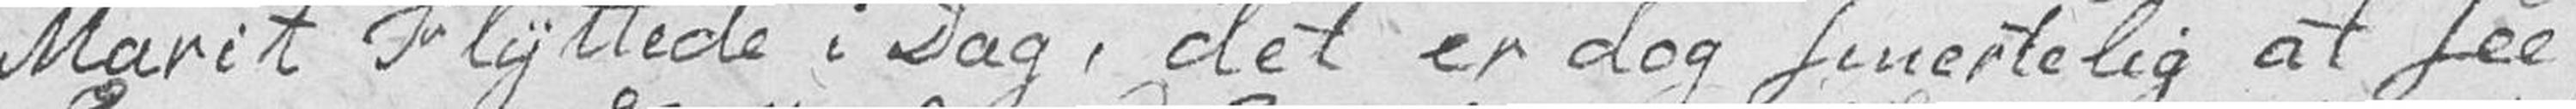Marit Flyttede i Dag, det er dog smertelig at see
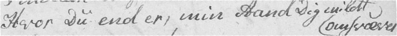Hvor Du end er, min Aand Dig mildt omsvæver
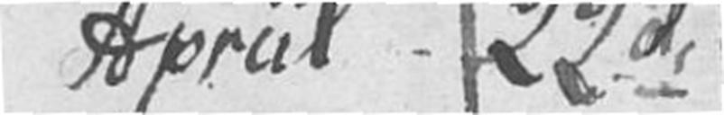Apriil 22d
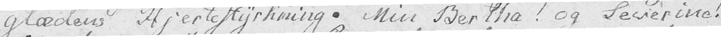glædens Hjertestyrkning. Min Bertha! og Severine!
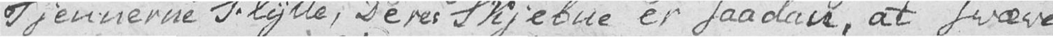Tjennerne Flytte, Deres Skjebne er saadan, at svæve
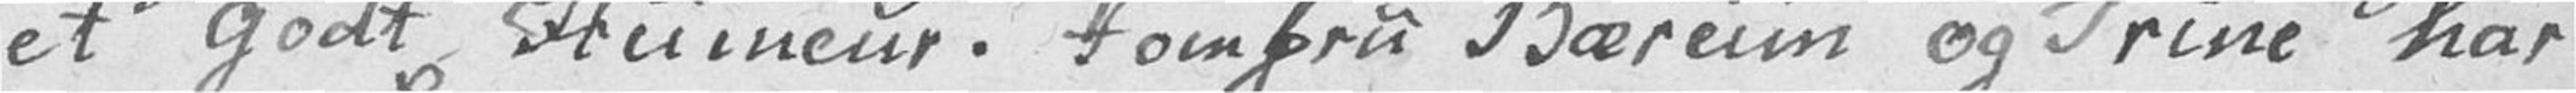et godt Humeur. Jomfru Bærum og Trine har
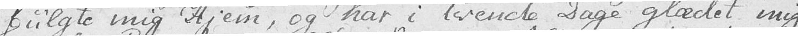fulgte mig Hjem, og har i tvende Dage glædet mig
